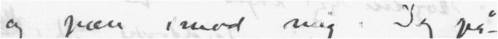og pæn imod mig. Jeg på-
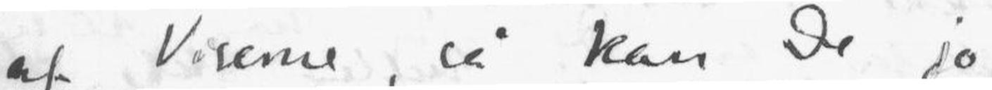af Viserne, så kan De jo
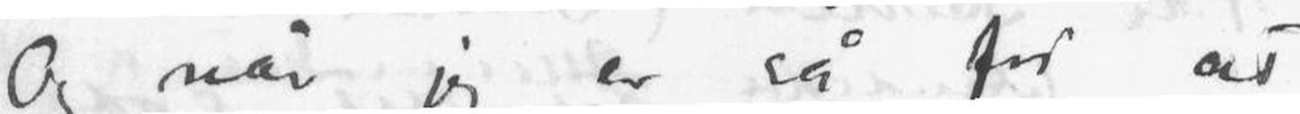Og når jeg er så fri at
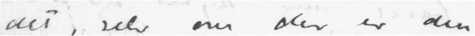det, selv om der er den
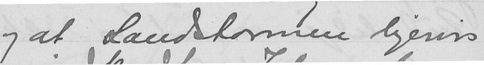og at Landstormen ligevis
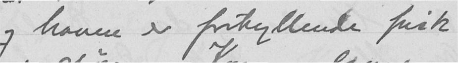og haven er fortryllende frisk
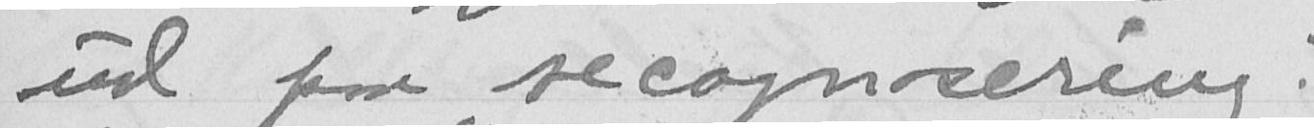ud paa "recognosering".
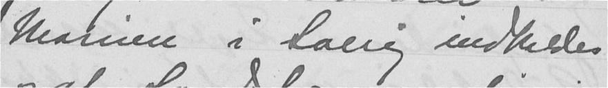Marinen i Sverig indkaldes
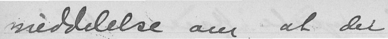meddelelse om, at der
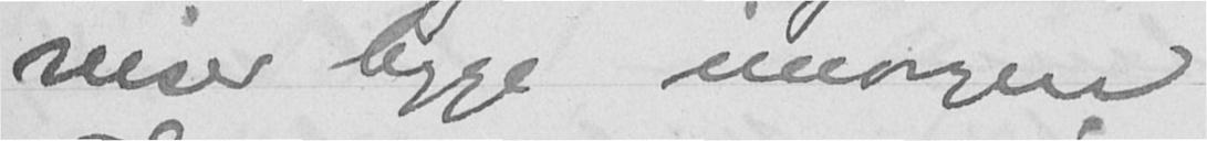reiser begge imorgen
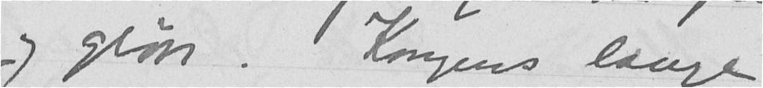og grøn. Kongens lange
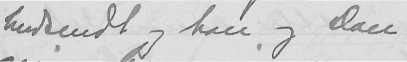budsendt og han og Don
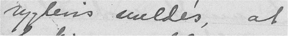rygtevis meldes, at
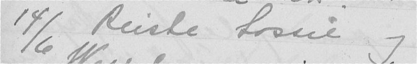14/6 Reiste Sossi og
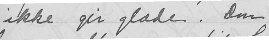ikke gir glæde. Don
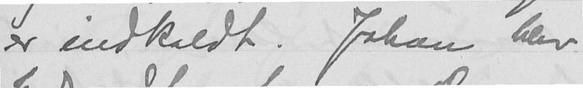er indkaldt. Johan blev
